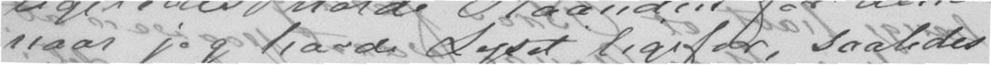naar jeg havde Lyset ligefor, saaledes
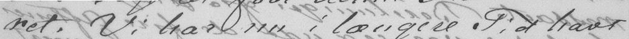ret. Vi har nu i længere Tid havt
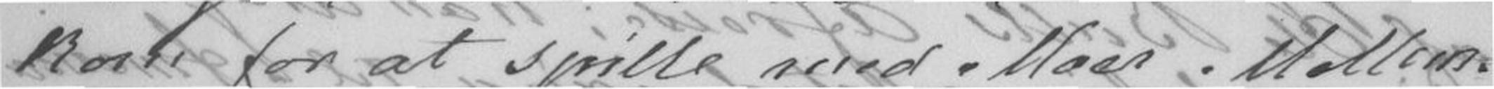kom for at spille med Moer Mellem-
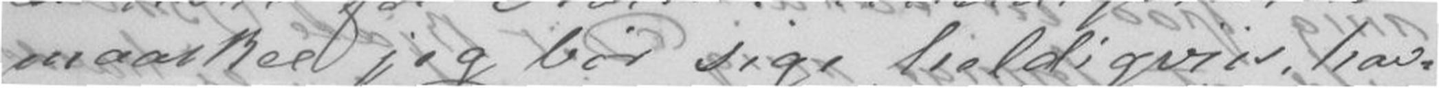maaskee jeg bør sige heldigviis hav-
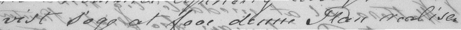vist søge at faae denne Plan realise-
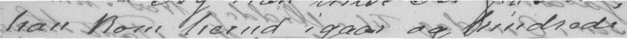han kom herud igaar og hindrede
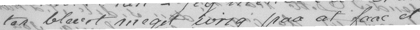ter blevet meget ivrig paa at faae et
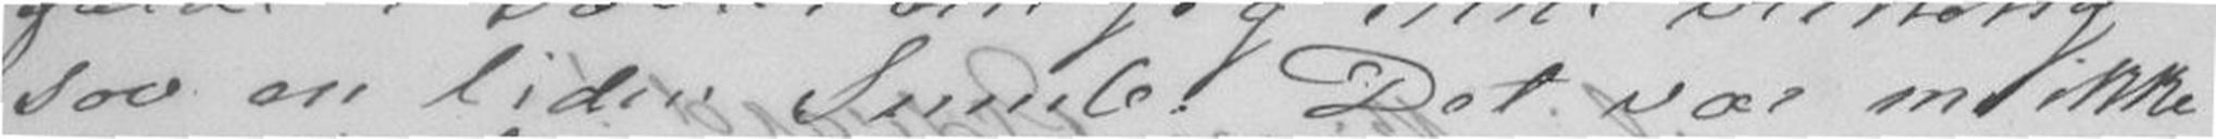sov en liden Smule. Det var nu ikke
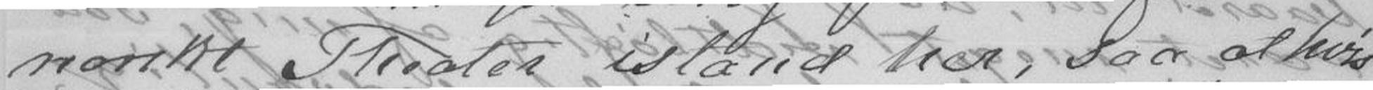norskt Theater istand her, saa at hvis
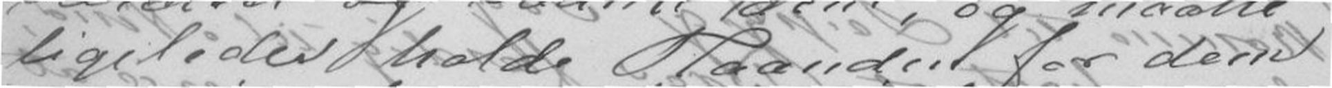ligeledes holde Haanden for dem
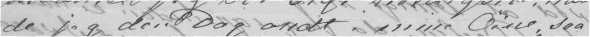de jeg den Dag ondt i mine Øine, saa
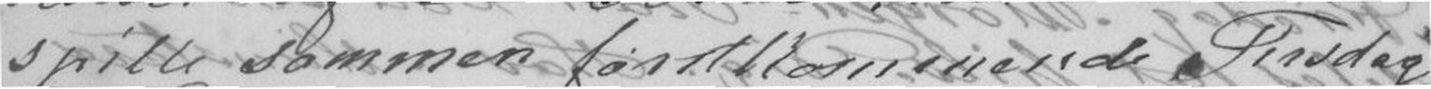spille sammen førstkommende Fredag
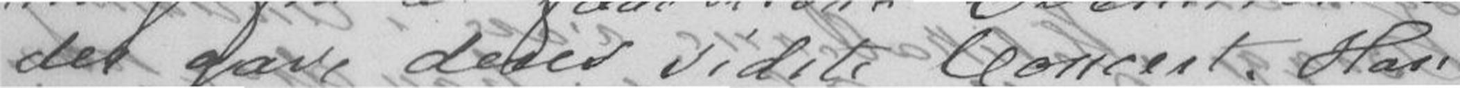der gave deres sidste Concert. Han
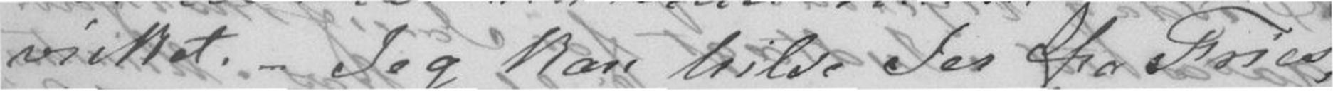virket. - Jeg kan hilse Jer fra Fries,
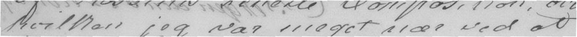hvilken jeg var meget nær ved at
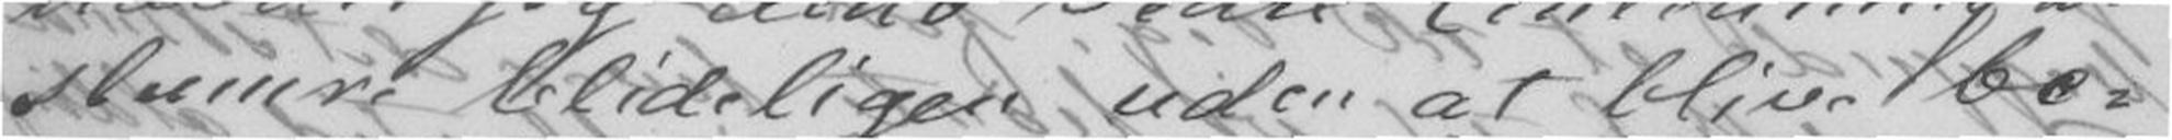slumme blideligen uden at blive be-
